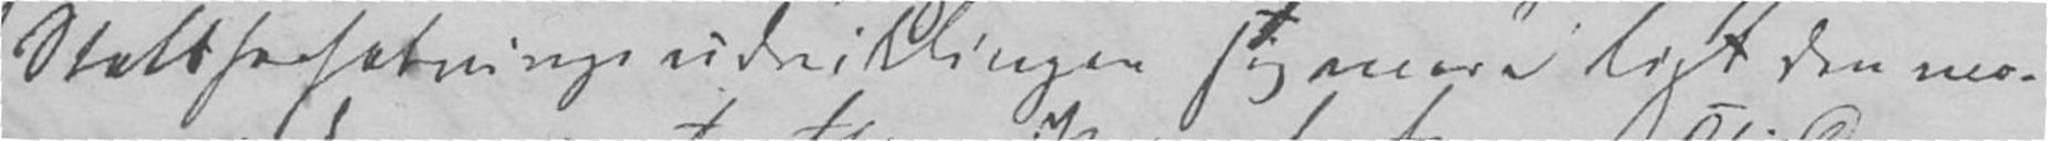Statsforfatningsudviklingen sig meere ligt den mo-
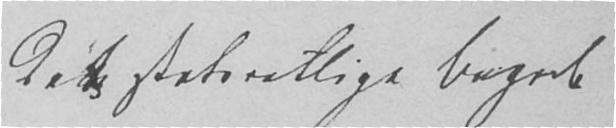det statsretlige Begreb
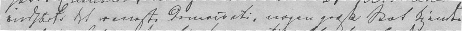indførte det reneste Democrati, nogen græsk Stat kjendte
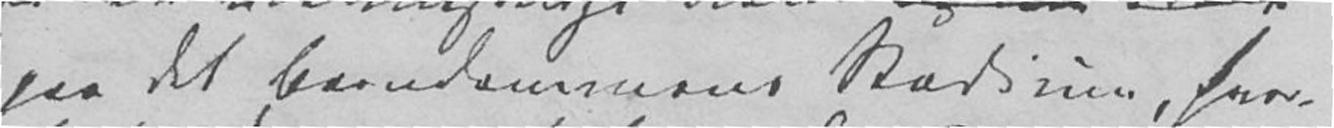paa det barndommens Stadium, hvor-
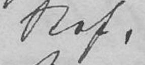Stof,
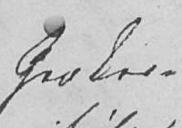Græker-
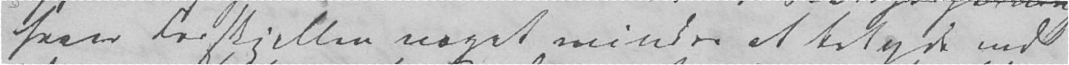faaer Forskjellen noget mindre at betyde end
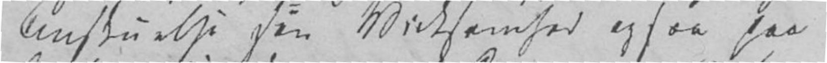Anskuelse sin Virksomhed ogsaa paa
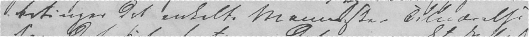betinger det enkelte Menneskes Tilværelse
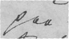paa
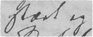stærk og
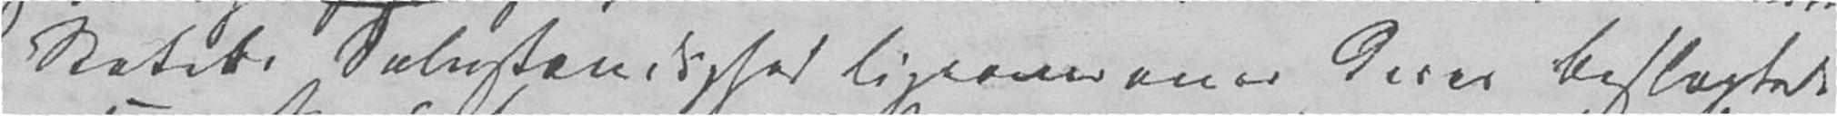Staters Selvstændighed ligeomener deres beslægtede
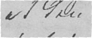at den
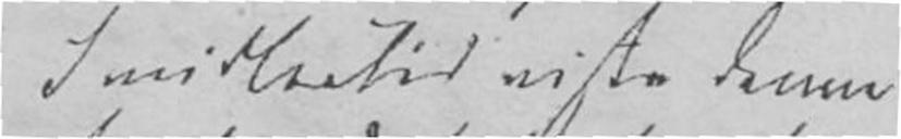Imidlertid viste denne
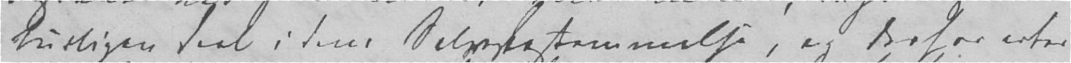turligen Deel i dens Selvbestemmelse, og derfor arter
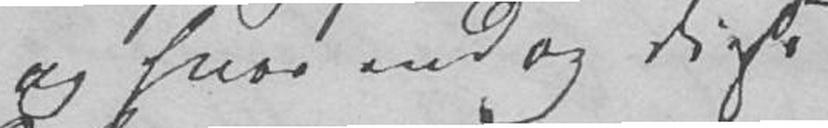og hvor endog disse
